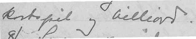kortspil og billiard.
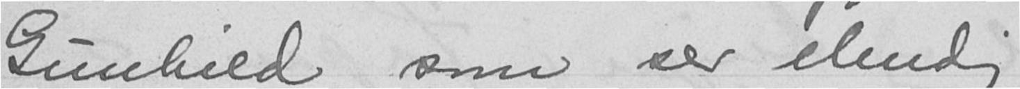Gunhild som ser elendig
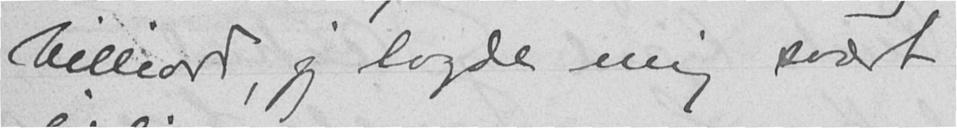billiard, jeg lagde mig svært
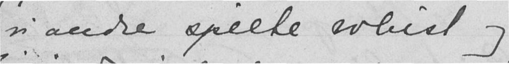vi andre spilte whist og
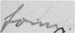form.
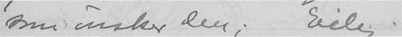som ønsker den; Eileg
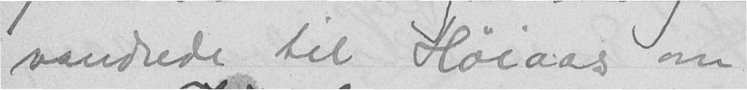vandrede til Høiaas om
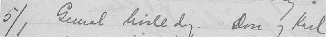5/1 Gemal hvile dag. Don og Karl
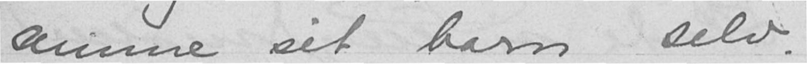amme sit barn selv.
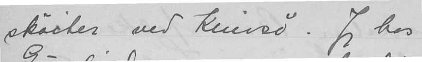skøiter ved Knivsø. Jeg hos
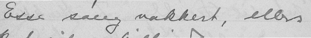Esse sang vakkert, ellers
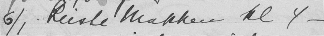6/1 Reiste Makken kl 4 -
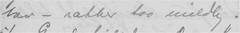barn - rather too wildly.
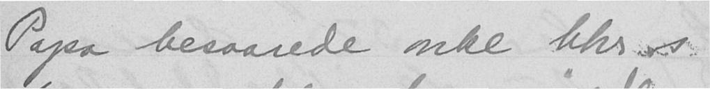Papa besaarede onkel Chr.s
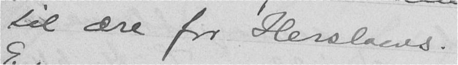til ære for Herslows.
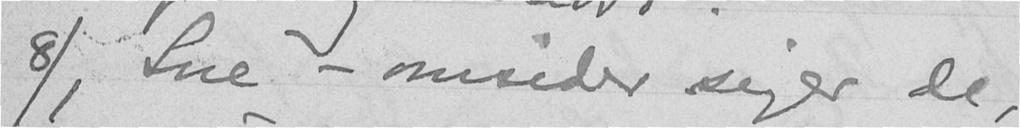8/1 Sne - omsider siger de,
